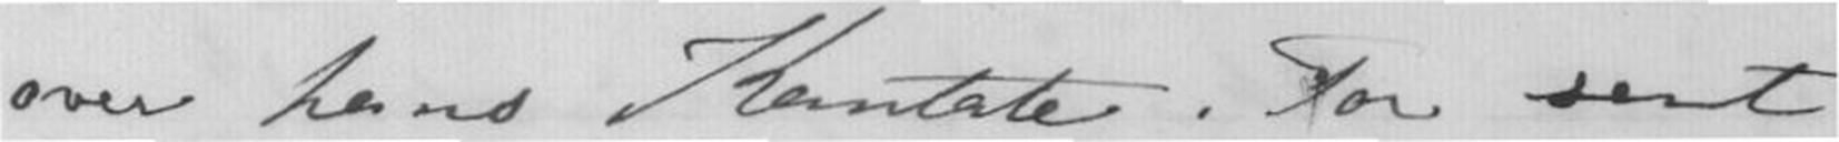over hans Kantate. For sent
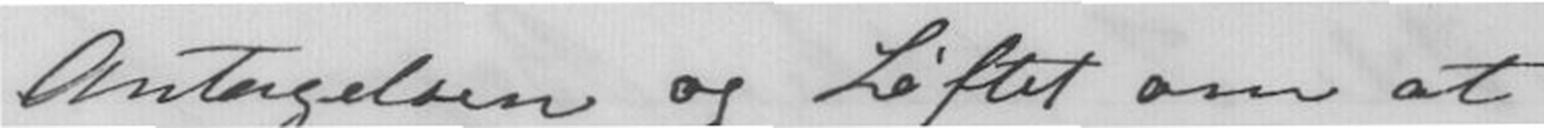Antagelsen og Løftet om at
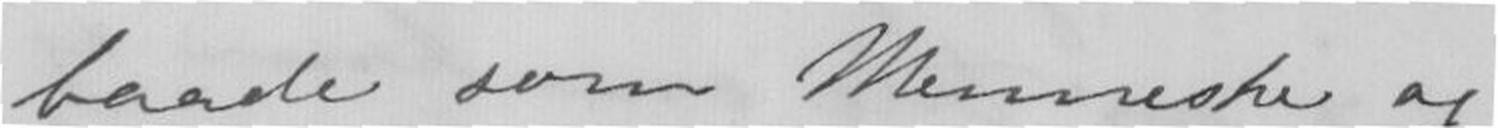baade som Menneske og
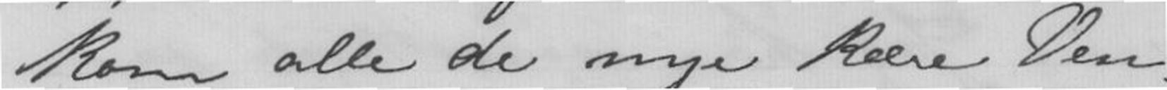kom alle de nye kære Ven-
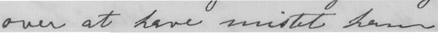over at have mistet ham
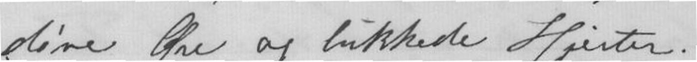døve Øre og lukkede Hjerter.
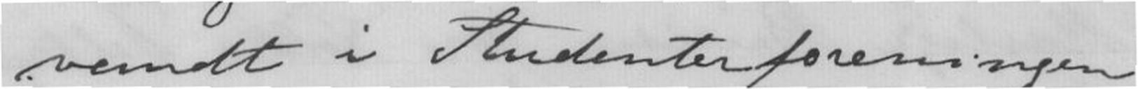vandt i Studenterforeningen
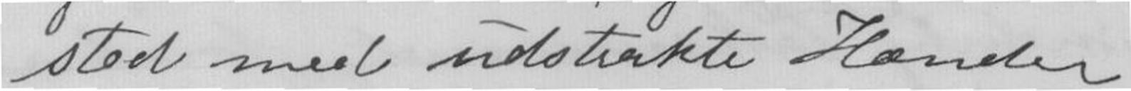stod med udstrakte Hænder
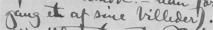gang et af sine billeder).
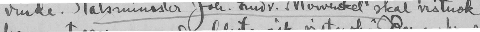vinde. Statsminister Joh. Ludv. Mowinckel skal vistnok
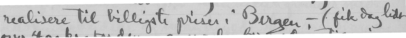realisere til billigste priser i Bergen, - (fik dog lidt
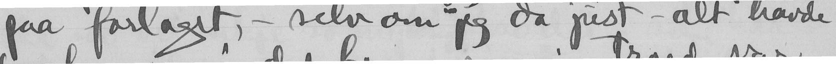paa forlaget, - selv om jeg da just - alt havde
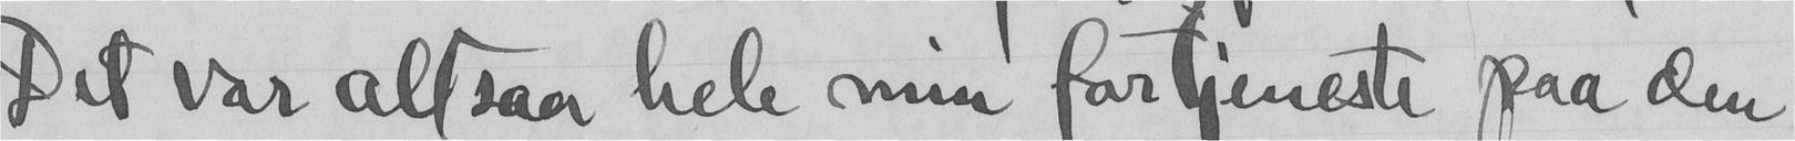Det var altsaa hele min fortjeneste paa den
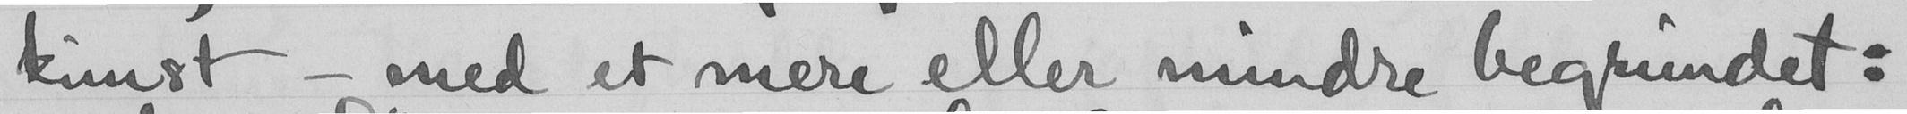kunst - med et mere eller mindre begrundet:
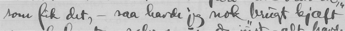som fik det, - saa havde jeg nok "brugt kjæft"
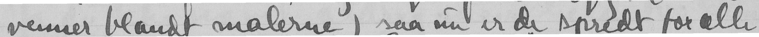venner blandt malerne) saa nu er de spredt for alle
